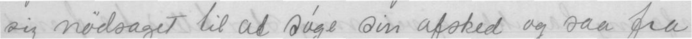sig nødsaget til at søge sin afsked og saa fra
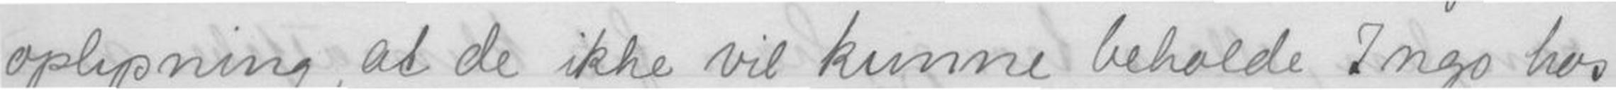oplysning, at de ikke vil kunne beholde Ingo hos
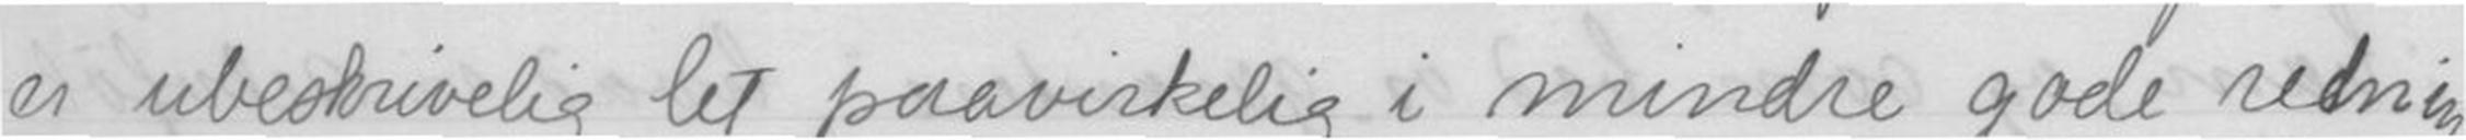er ubeskrivelig let paavirkelig i mindre gode retnin-
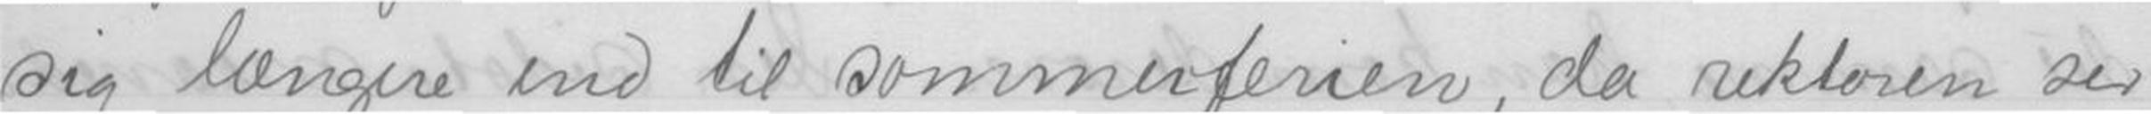sig længere end til sommerferien, da rektoren ser
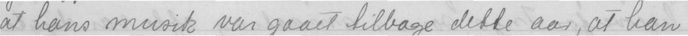at hans musik var gaaet tilbage dette aar, at han
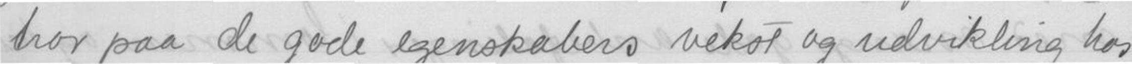tror paa de gode egenskabers vekst og udvikling hos
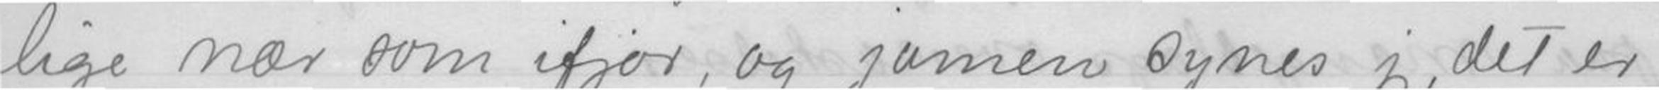lige nær som ifjor, og jammen synes jeg, det er
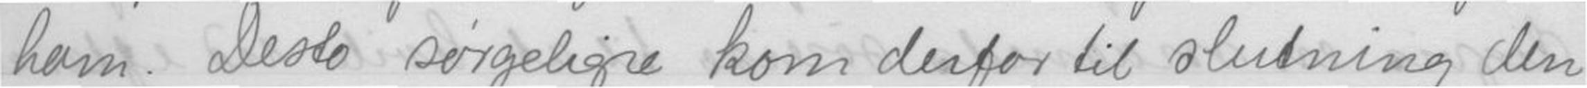ham. Desto sørgeligre kom derfor til slutning den
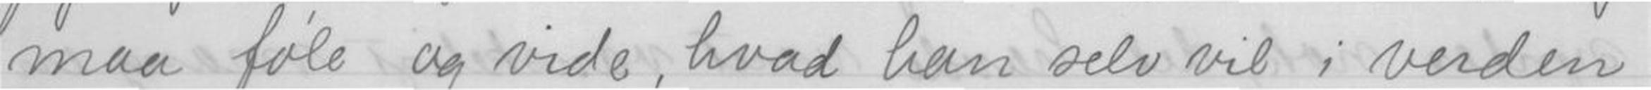maa føle og vide, hvad han selv vil i verden
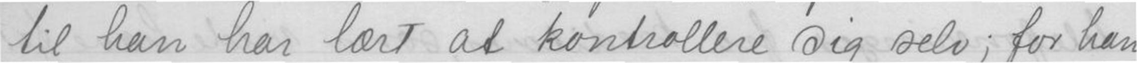til han har lært at kontrollere sig selv; for han
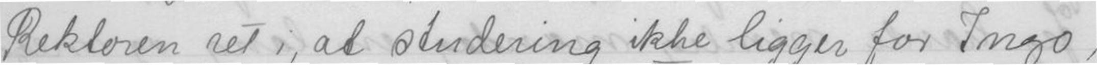Rektoren ret i, at studering ikke ligger for Ingo,
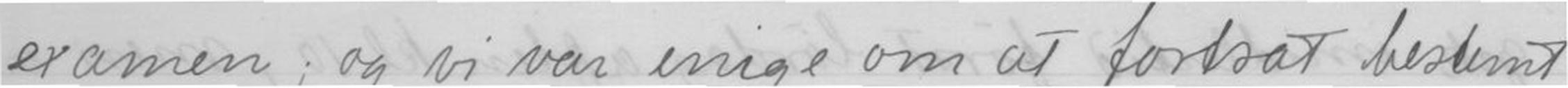examen; og vi var enige om at fortsat bestemt
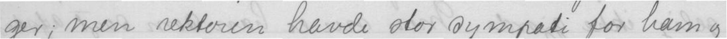ger; men rektoren havde stor sympati for ham og
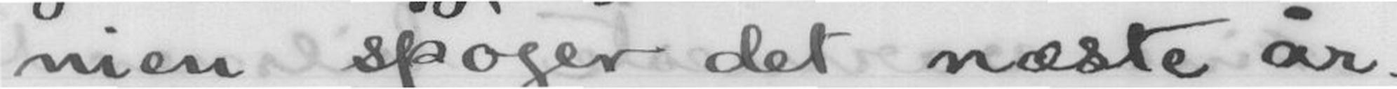nien spøger det næste år.
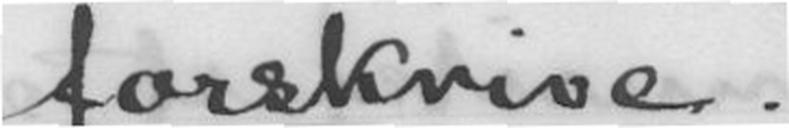forskrive.
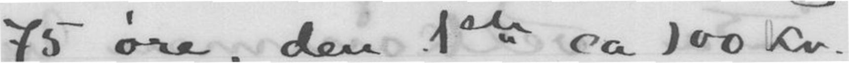75 øre, den 1ste ca 100 kr.
